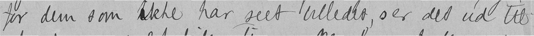for dem som ikke har seet billedet, ser det ud til
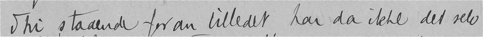Thi staaende foran billedet, har da ikke det selv
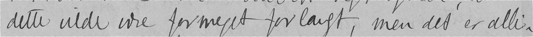dette vilde være formeget forlangt, men det er alli-
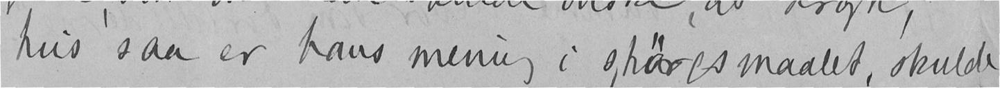hvis saa er hans mening i spørgsmaalet, skulde
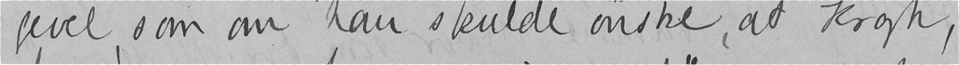gevel, som om han skulde ønske, at Krogh,
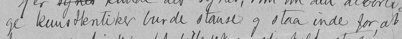ge kunstkritiker burde stanse og staa inde for, at
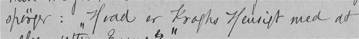spørger. "Hvad er Kroghs Hensigt med at
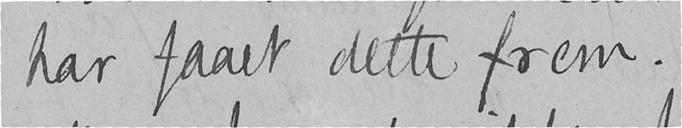har faaet dette frem.
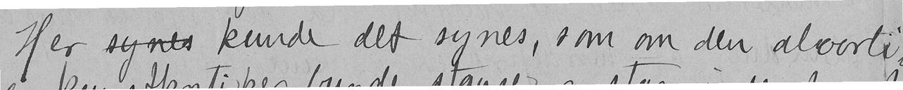Her synes kunde det synes, som om den alvorli-
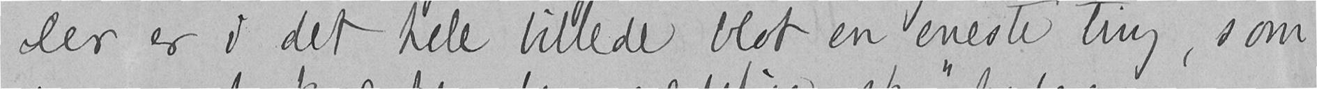Der er i det hele billede blot en eneste ting, som
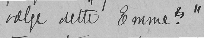vælge dette Emme?"
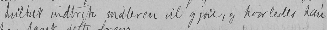hvilket indtryk maleren vil gjøre, og hvorledes han
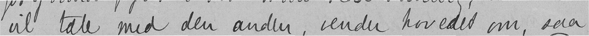vil tale med den anden, vender hovedet om, saa
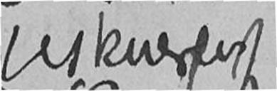beskuerlet
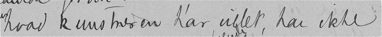hvad kunstneren har villet, har ikke
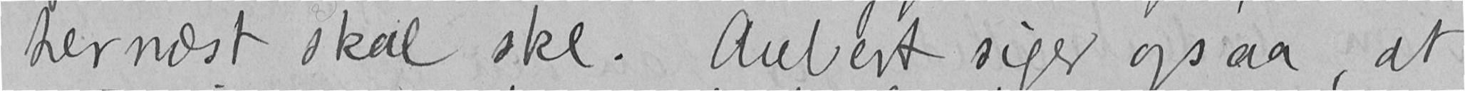hernæst skal ske. Aubert siger ogsaa, at
